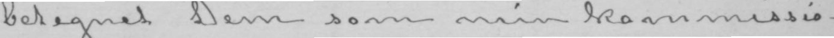betegnet Dem som min kommissio-
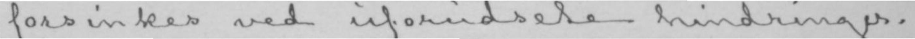forsinkes ved uforudsete hindringer.
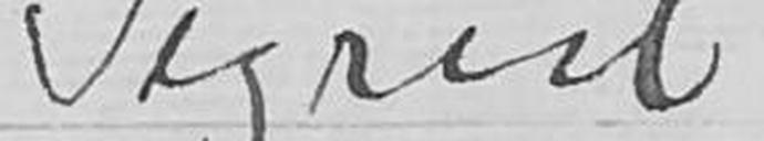Sigrid
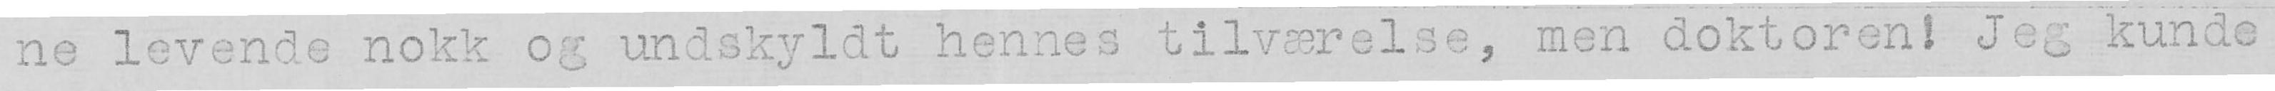ne levende nokk og undskyldt hennes tilværelse, men doktoren! jeg kunde,
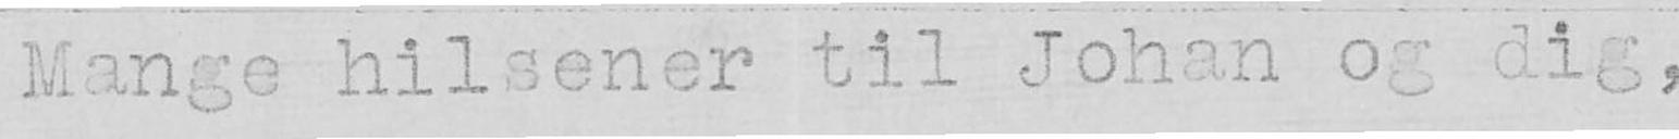Mange hilsener til Johan og dig,
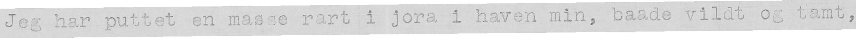Jeg har puttet en masse rart i jora i haven min, baade vildt og tamt,
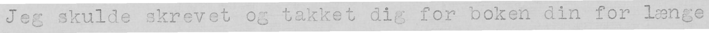Jeg skulde skrevet og takket dig for boken din for længe
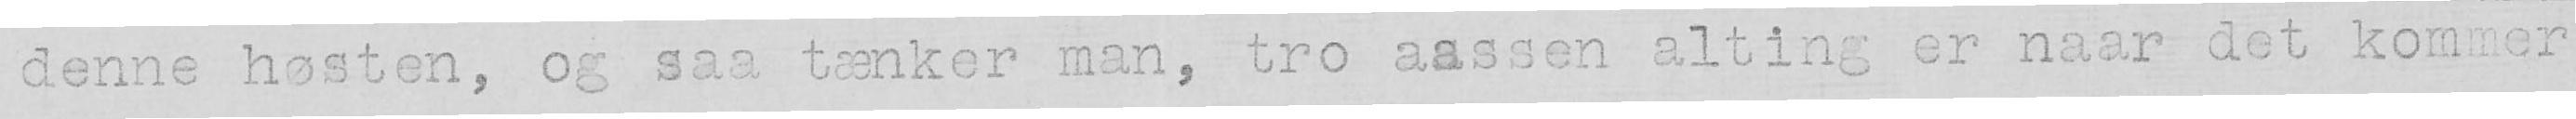denne høsten, og saa tænker man, tro aassen alting er naar det kommer
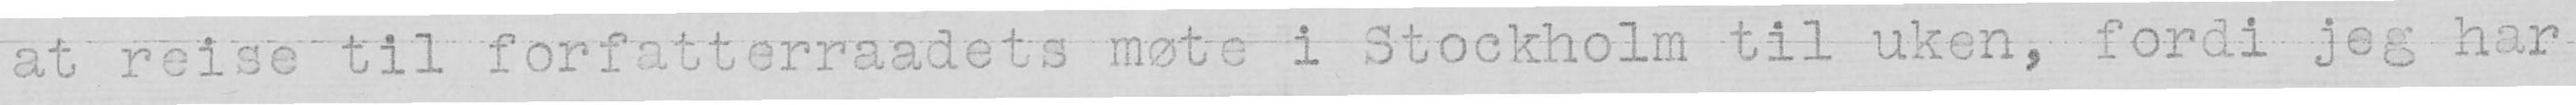at reise til forfatterraadets møte i Stockholm til uken, fordi jeg har
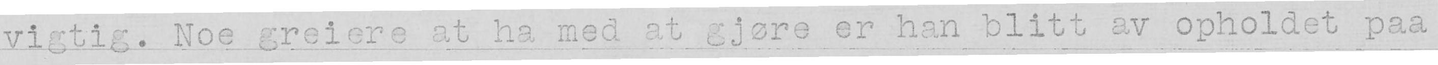vigtig. Noe greiere at ha med at gjøre er han blitt av opholdet paa
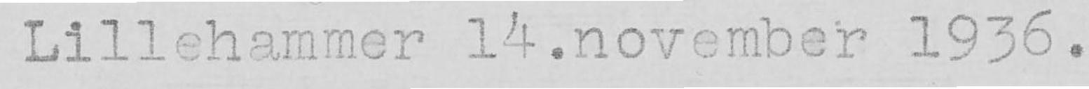Lillehammer 14. november 1936.
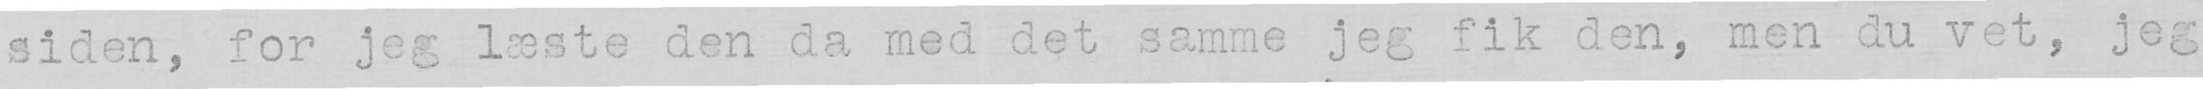siden, for jeg læste den da med det samme jeg fik den, men du vet, jeg
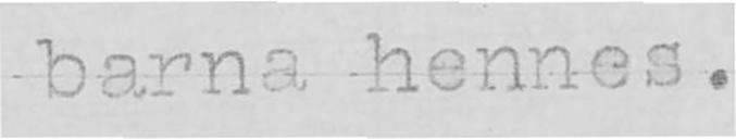barna hennes.
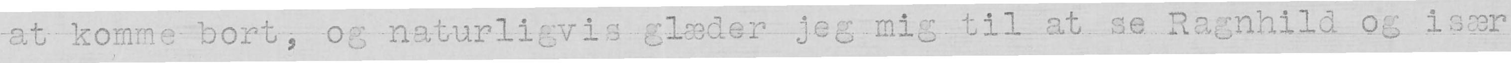at komme bort, og naturligvis glæder jeg mig til at se Ragnhild og især
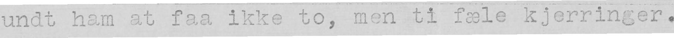undt ham at faa ikke to, men ti fæle kjerringer.
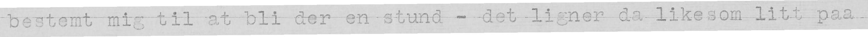bestemt mig til at bli der en stund – det ligner da likesom litt paa
